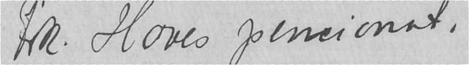Frk. Hoves pensionat,
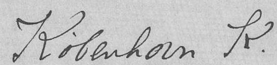København K.
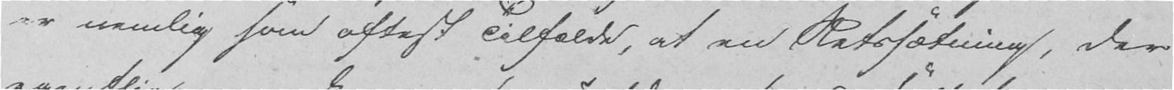er nemlig som oftest Tilfælde, at en Retssætning, der
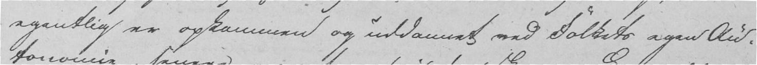egentlig er opkommen og uddannet ved Folkets egen Au-
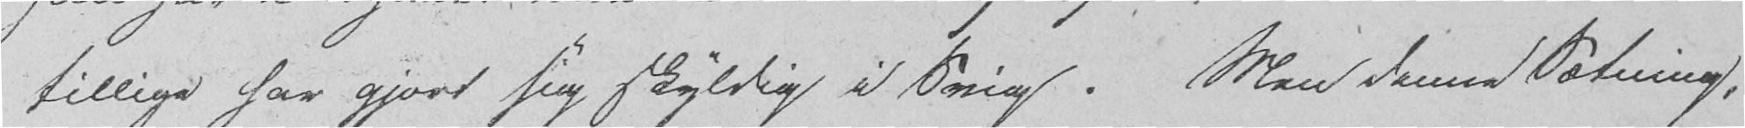tillige har gjort sig skyldig i Svig. Men denne Sætning,
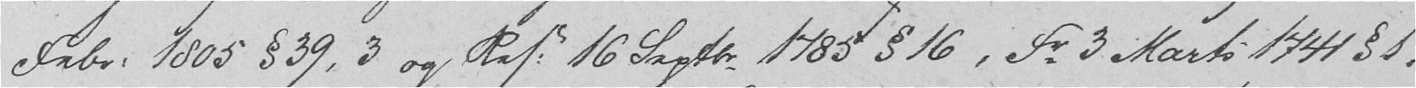Febr. 1805 § 39, 3 og Res. 16 Septr. 1785 § 16, Fr 3 Marti 1741 § 1,
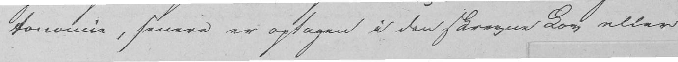tonomie, senere er optagen i den skrevne Lov eller
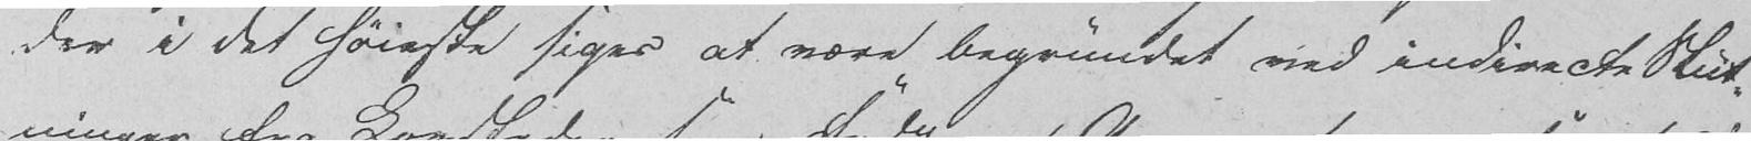der i det høieste siges at være begrundet ved indirecte Slut-
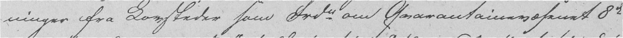ninger fra Lovsteder som Frdn om Qvarantainevæsenet 8de
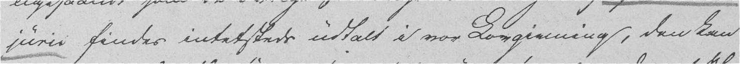juris findes intetsteds udtalt i vor Lovgivning, den kan
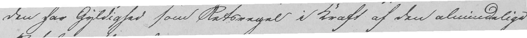den har Gyldighed som Retsregel i kraft af den almindelige
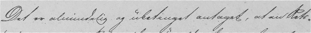Det er almindelig og ubetinget antaget, at en Rets-
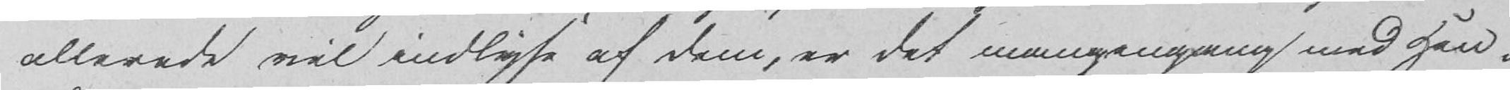allerede vil indlyse af dem, er det mangengang med Hen-
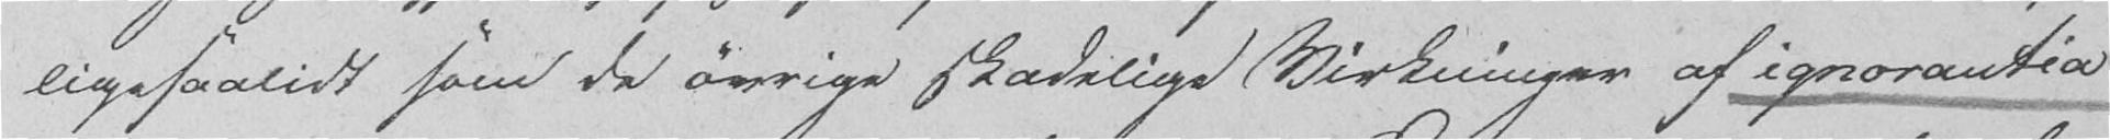ligesaalidt som de øvrige skadelige Virkninger af ignorantia
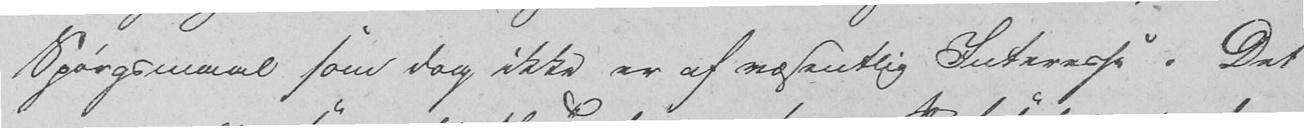Spørgsmaal som dog ikke er af væsentlig Interesse. Det
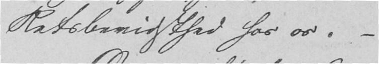Retsbevidsthed hos os. -
